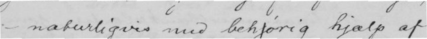- naturligvis med behørig hjælp af
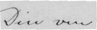Din ven
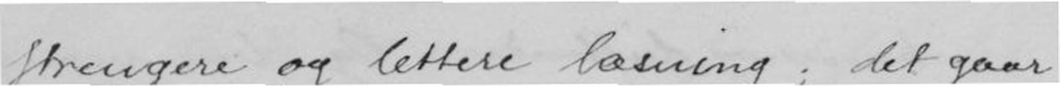strengere og lettere læsning; det gaar
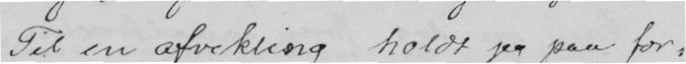Til en afvikling holdt jeg paa for-
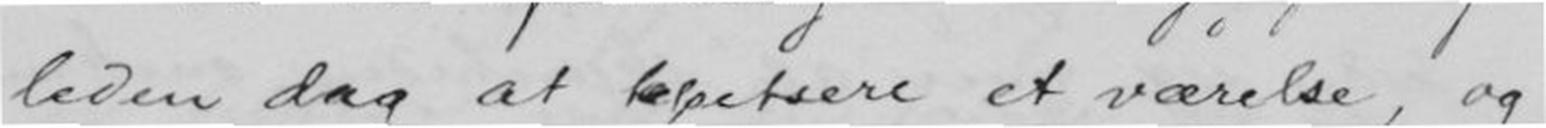leden dag at tapetsere et værelse, og
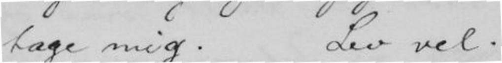tage mig. Lev vel.
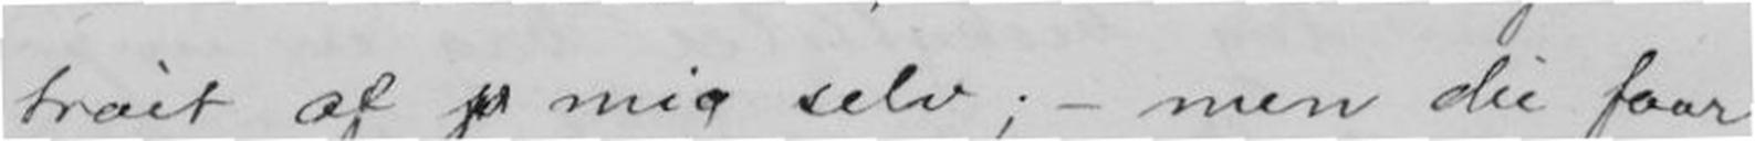trait af mig selv; - men du faar
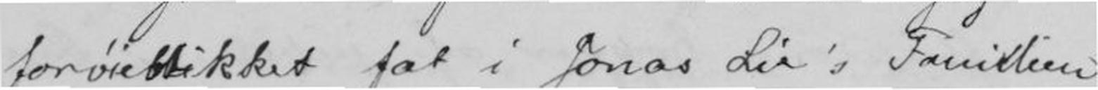forøieblikket fat i Jonas Lie's Familien
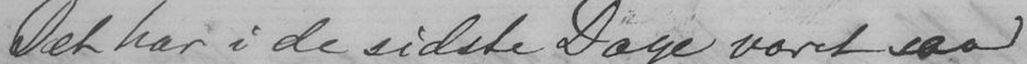Det har i sidste Dage været saa
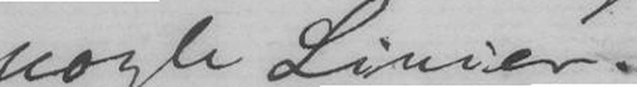nogle Linier.
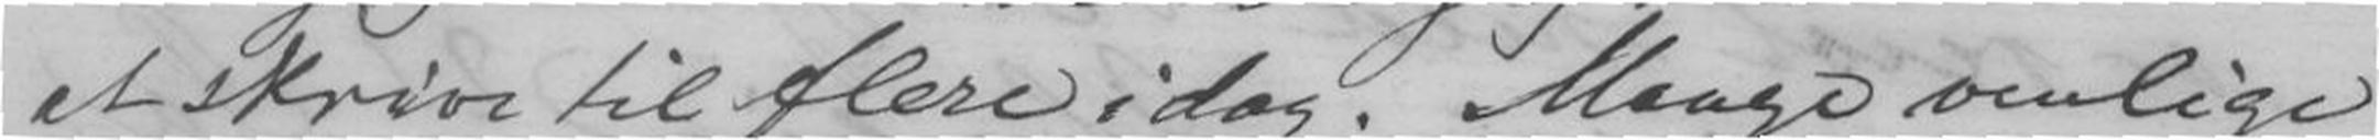at skrive til flere i dag. Mange venlige
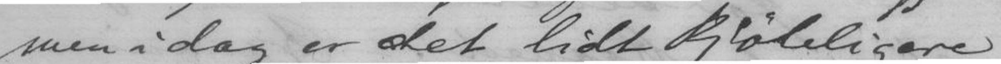men i dag er det lidt kjøligere
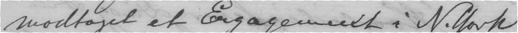modtaget et Engagement i N. York
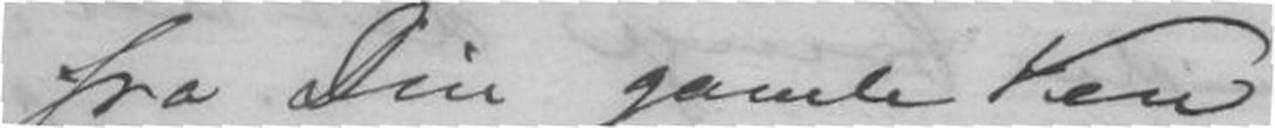fra Din gamle Ven
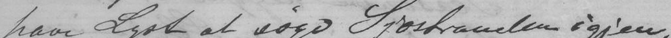have Lyst at søge Sjøstranden igjen,
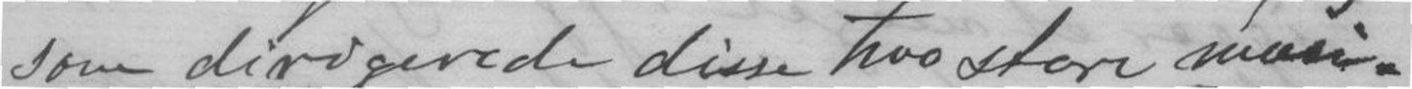som dirigerede disse two store musi-
